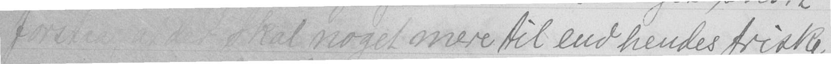forstaa, at der skal noget mere til end hendes friske
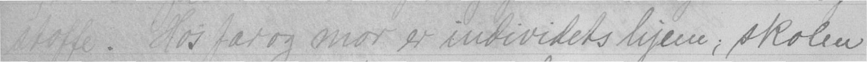stoffe. Hos far og mor er individets hjem; skolen
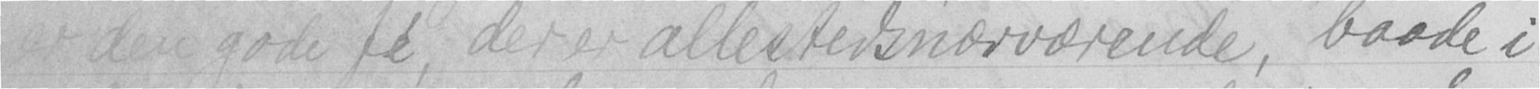er den gode fe, der er allestedsnærværende, baade i
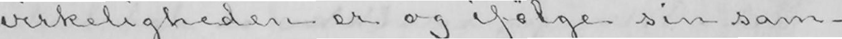virkeligheden er og ifølge sin sam-
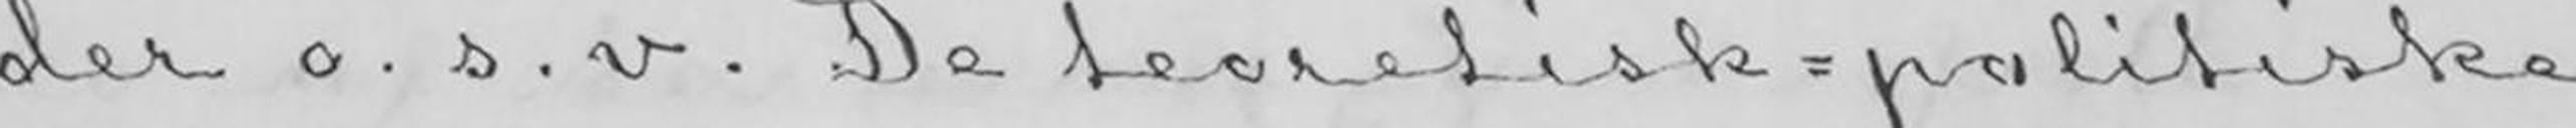der o. s. v. De teoretisk-politiske
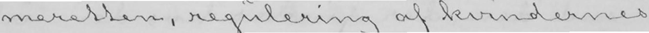meretten, regulering af kvindernes
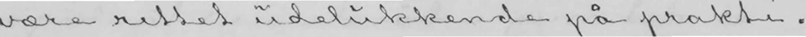være rettet udelukkende på prakti-
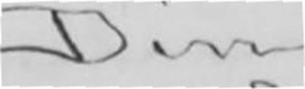Din
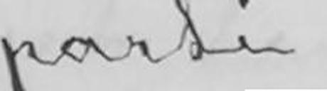parti.
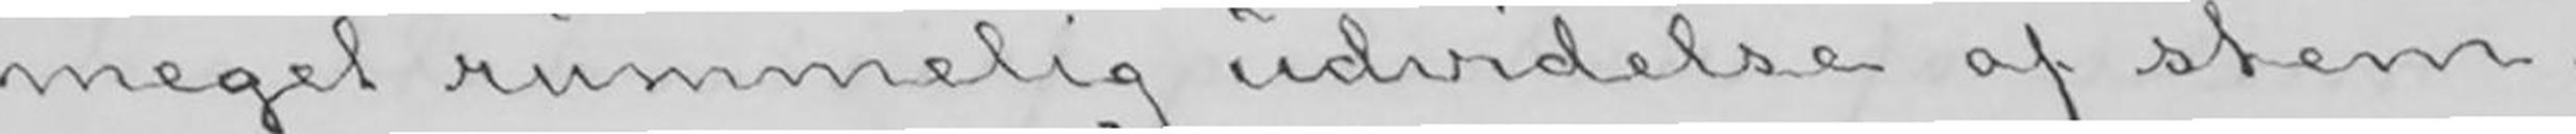meget rummelig udvidelse af stem-
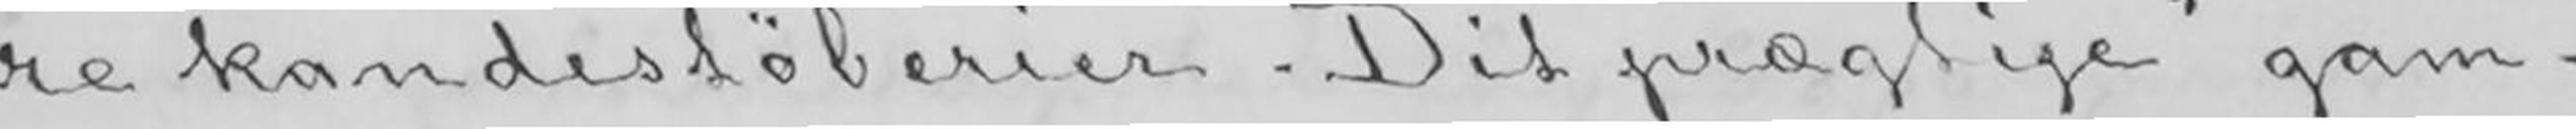re kandestøberier. Dit prægtige «gam-
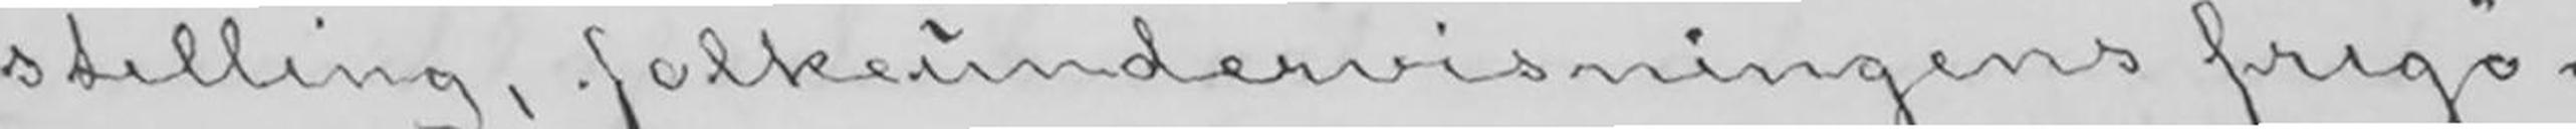stilling, folkeundervisningens frigø-
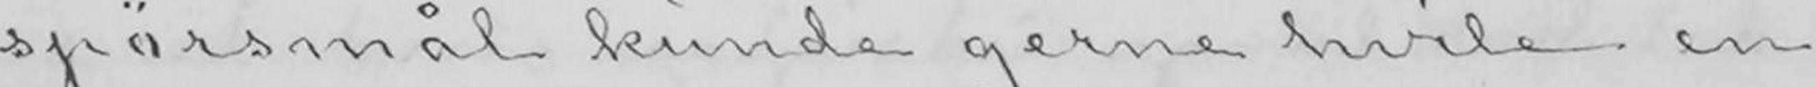spørsmål kunde gerne hvile en
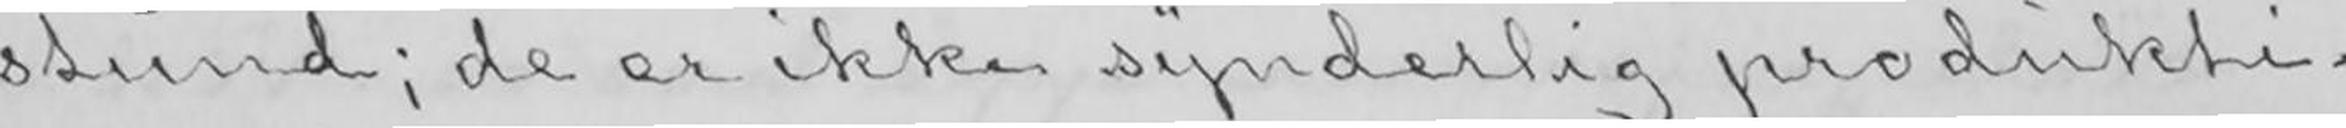stund; de er ikke synderlig produkti-
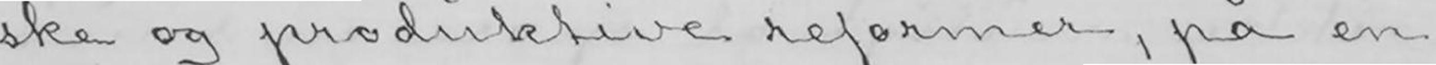ske og produktive reformer, på en
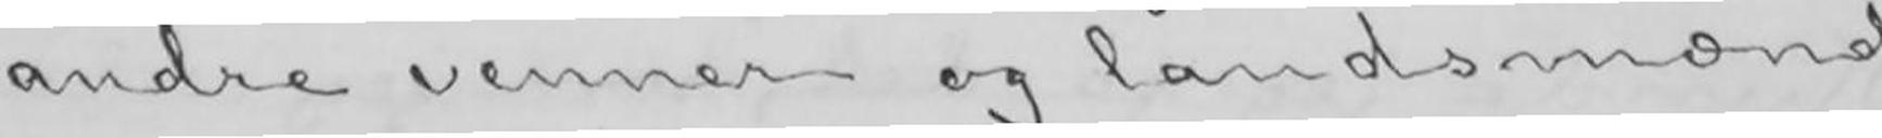andre venner og landsmænd.
